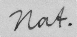Nat.
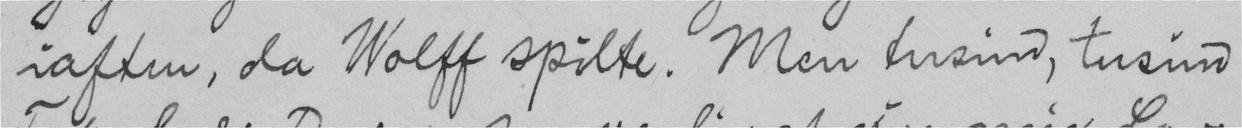iaften, da Wolff spilte. Men tusind, tusind
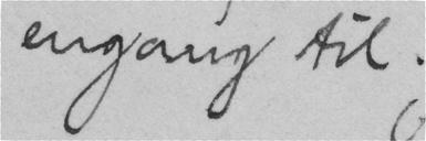engang til.
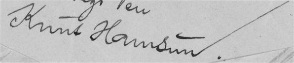Knut Hamsun.
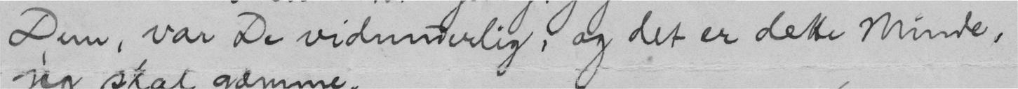Dem, var De vidunderlig, og det er dette Minde,
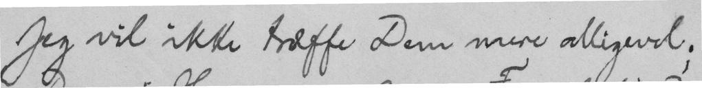Jeg vil ikke træffe Dem mere alligevel;
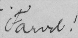Farvel!
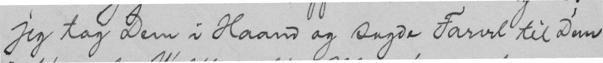jeg tog Dem i Haand og sagde Farvel til Dem
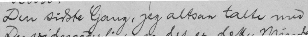Den sidste Gang, jeg altsaa talte med
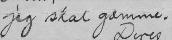jeg skal gæmme.
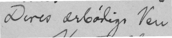Deres ærbødige Ven
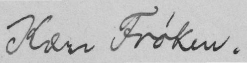Kære Frøken.
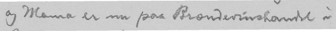og Mama er nu paa Brændevinshandel i
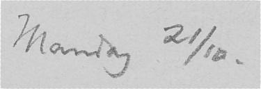Mandag 21/10.
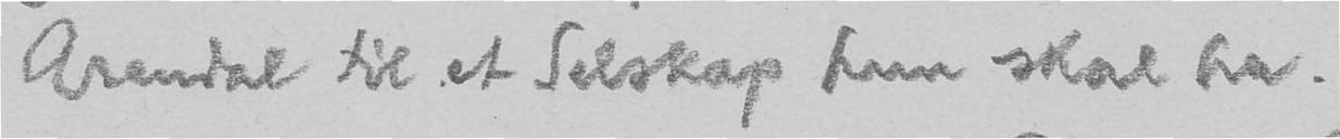Arendal til et Selskap hun skal ha.
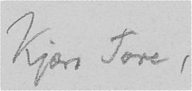Kjære Tore,
